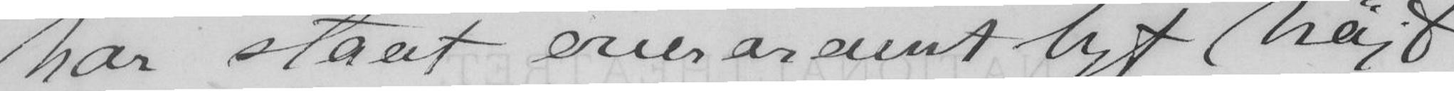har staat overordentligt højt.
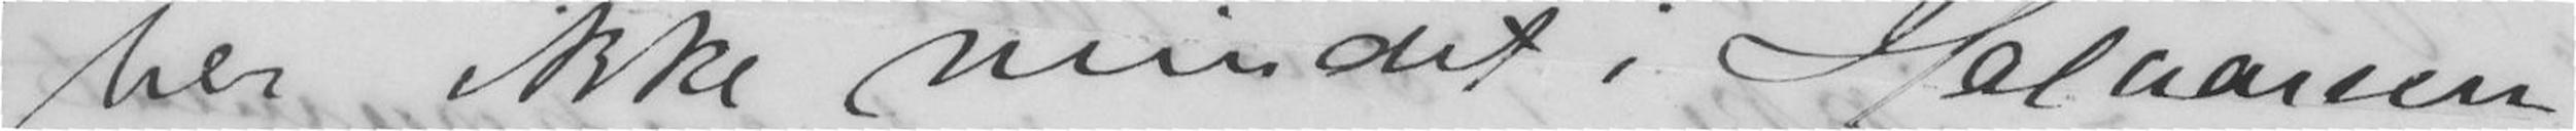her ikke mindet i Halvorsen -
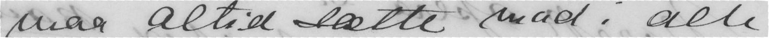Jeg maa altid sætte mod i alle
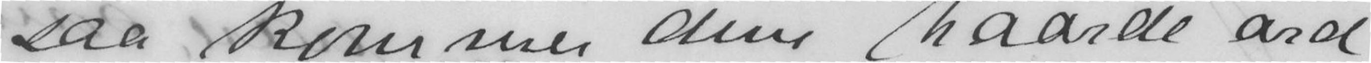saa vi kommer dine haarde ord.
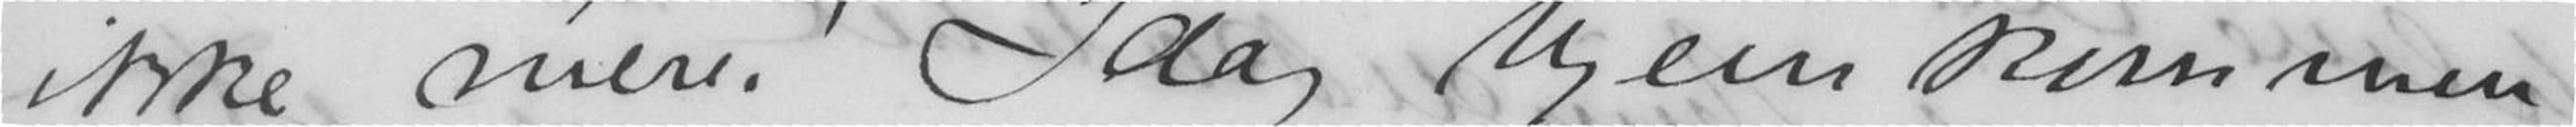ikke mere! Idag hjem kommen
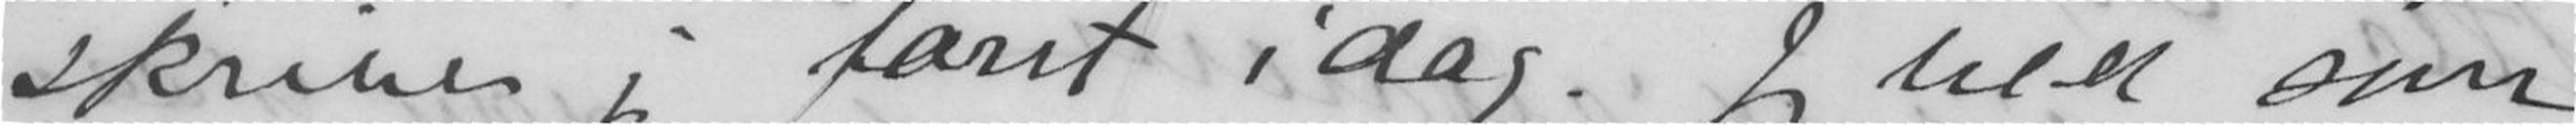skriver jeg først idag. Jeg ved som
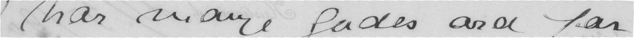Jeg har mange Gudes ord for
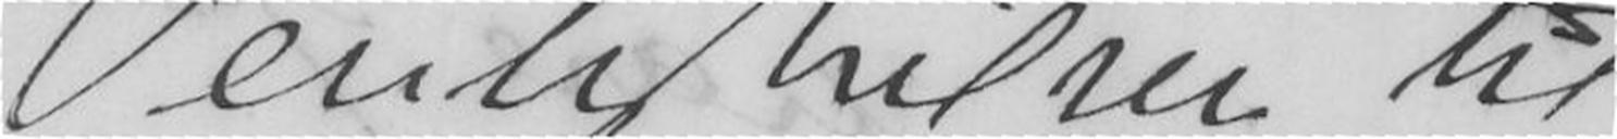Venlig hilsen til
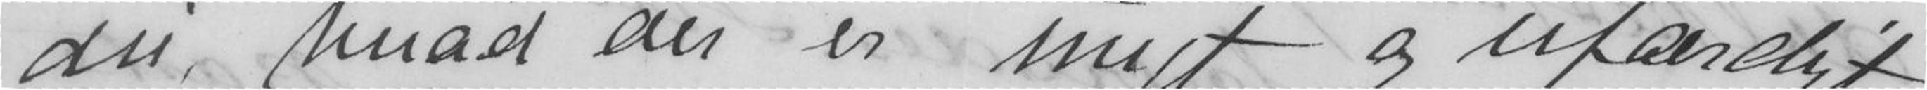du, hvad der er ungt og ufærdigt;
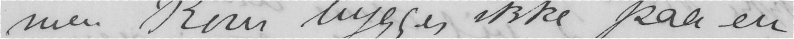men Rom bygges ikke paa en
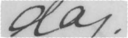dag!
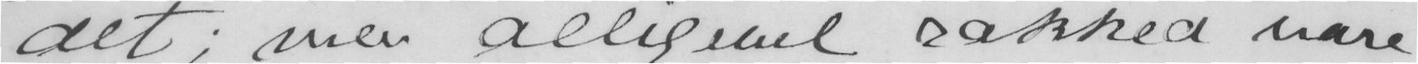det; men alligevel rakked vore
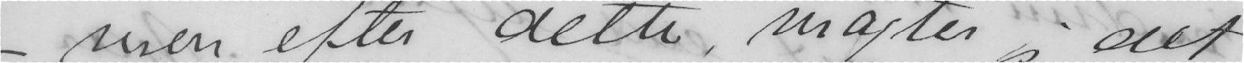men efter dette magter jeg det
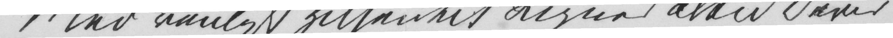Med venligst Hilsen til Sigurd altid Deres
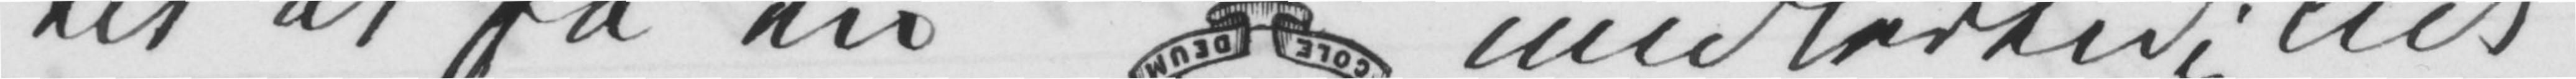til at få en midlertidig lidt
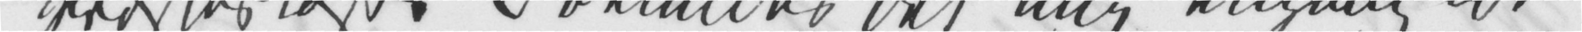trøstesløst. Forundes det mig engang at
